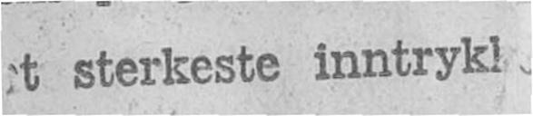t sterkeste inntryk
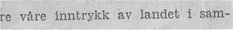re våre inntrykk av landet i sam-
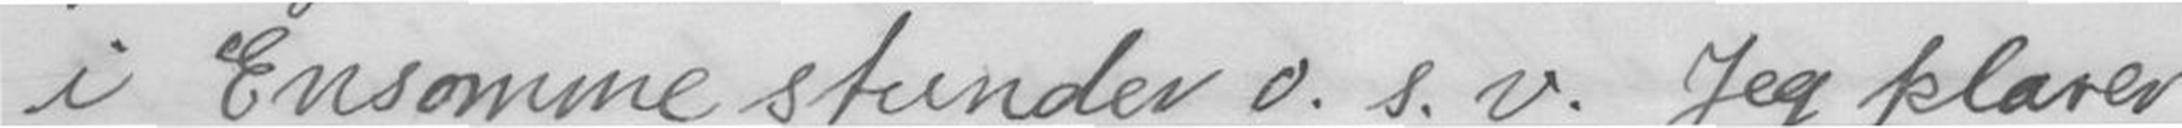i Ensomme stunder o.s.v. Jeg klarer
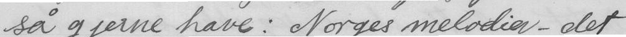så gjerne have: Norges melodier - det
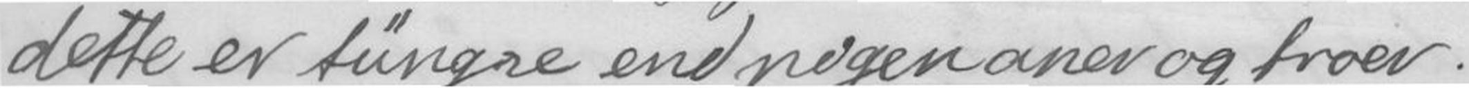dette er tüngre end nogen aner og troer.
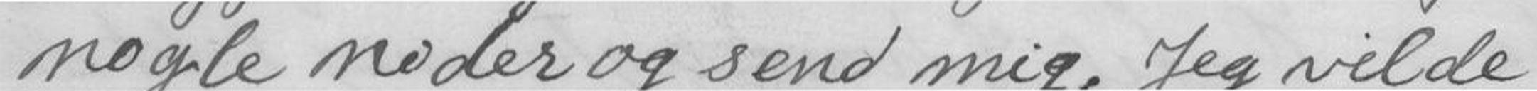nogle noder og send mig. Jeg vilde
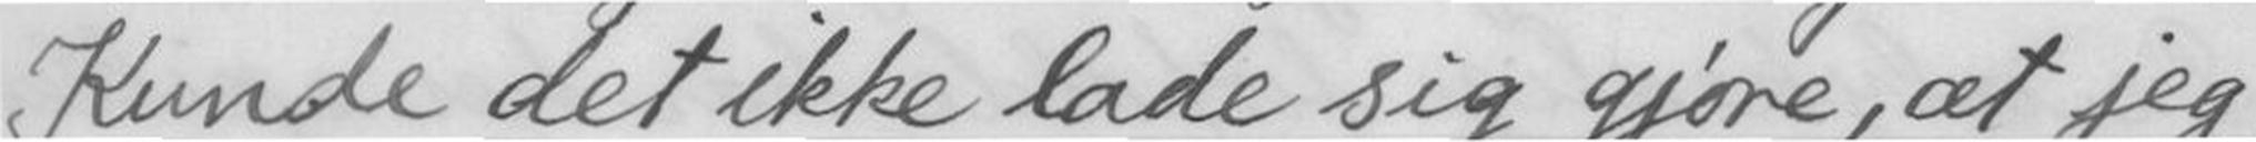Kunde det ikke lade sig gjøre, at jeg
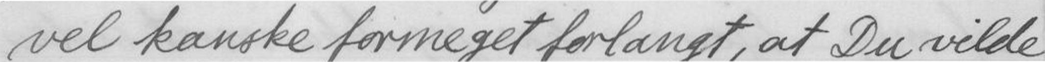vel kanske formeget forlangt, at Du vilde
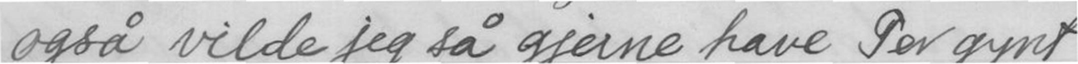også vilde jeg så gjerne have Per gynt
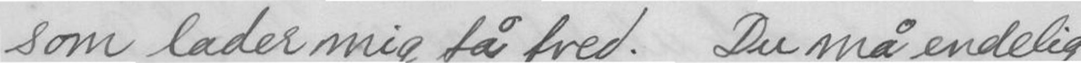som lader mig få fred. Du må endelig
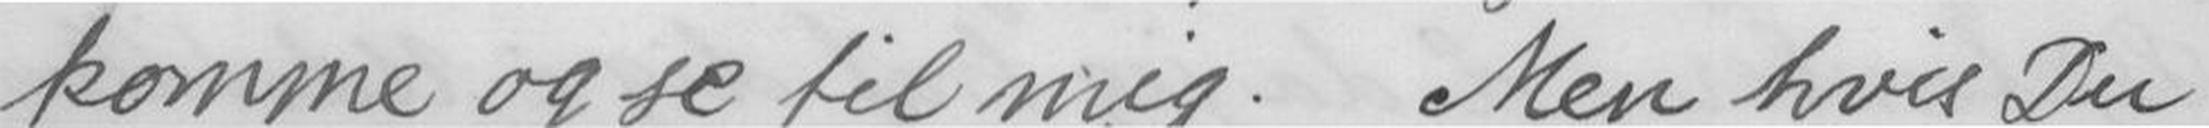komme og se til mig. Men hvis Du
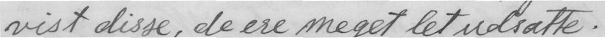vist disse, de ere meget let udsatte.
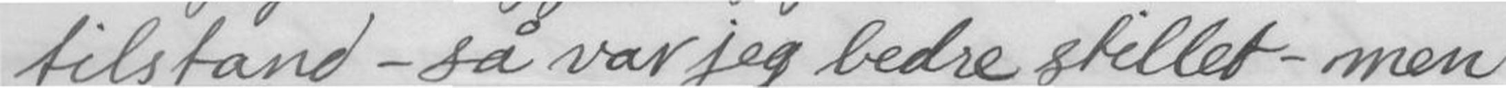tilstand - så var jeg bedre stillet - men
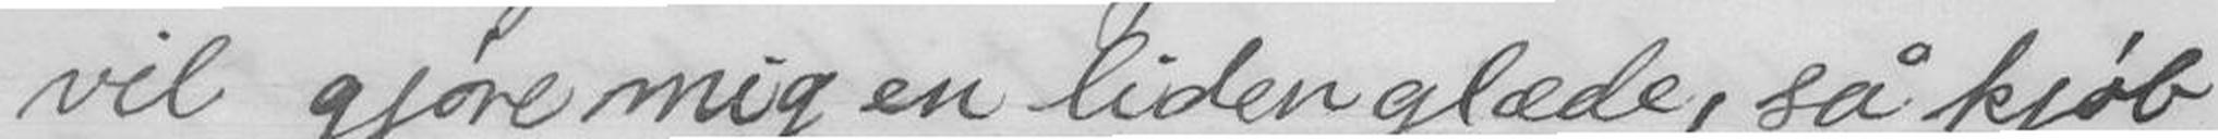vil gjøre mig en liden glæde, så kjøb
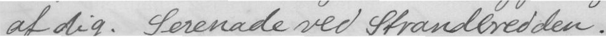af dig. Serenade ved Strandbredden.
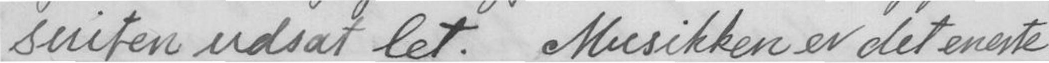suiten udsat let. Musikken er det eneste
